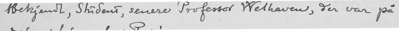Bekjendt, Student, senere Professor Welhaven, der var på
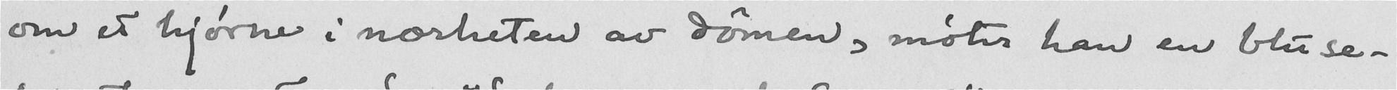om et hjørne i nærheten av dømen, møter han en bluse-
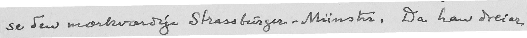se den mærkværdige Strassburger-Münster. Da han dreier
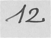12
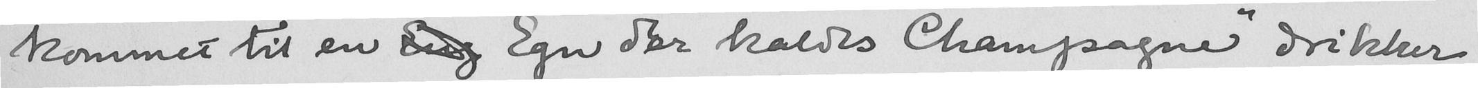kommet til en Eng Egn der kaldes Champagne" drikker
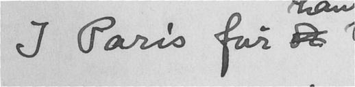I Paris får d
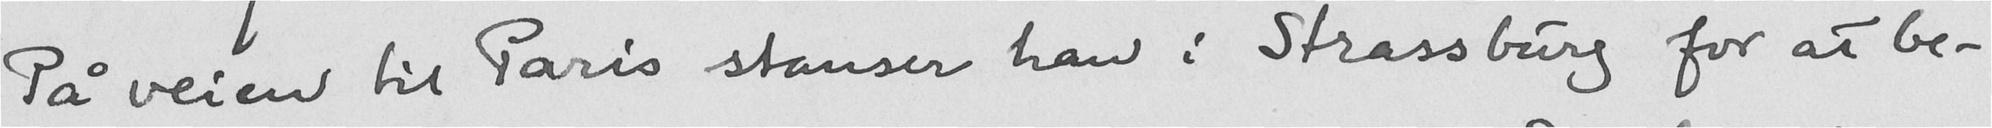På veien til Paris stanser han i Strassburg for at be-
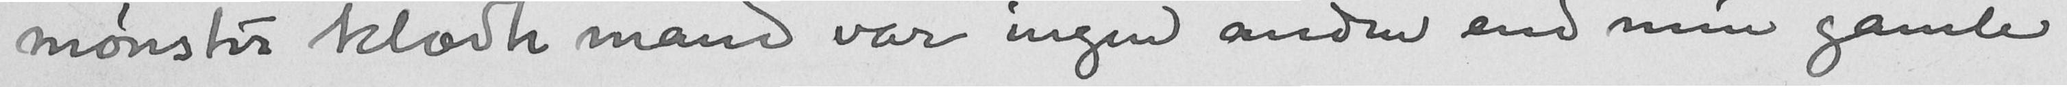mønster klædte mand var ingen anden end min gamle
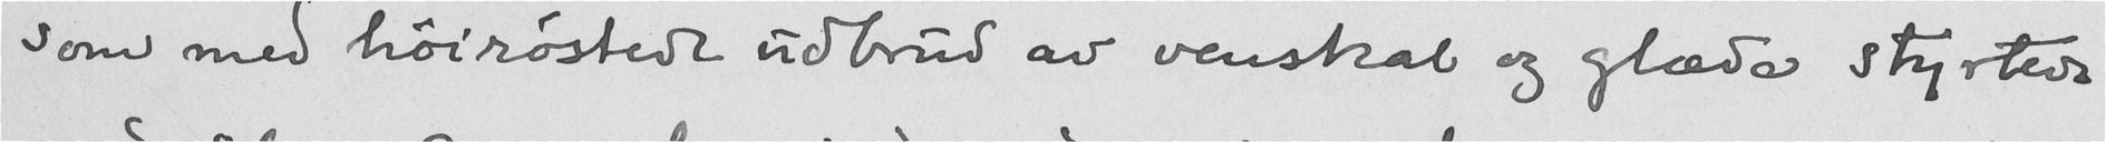som med høirøstede udbrud av venskab og glæde styrtede
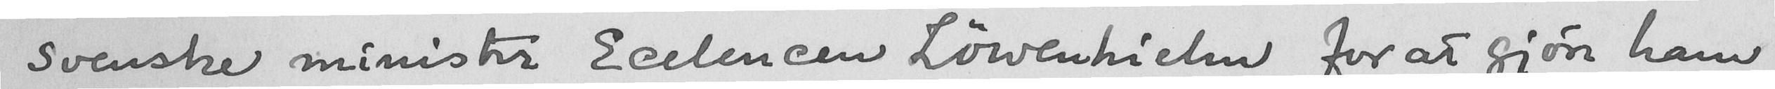svenske minister Ecelencen Løwenhielm for at gjøre ham
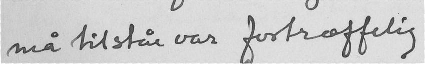må tilståe var fortræffelig"
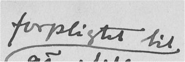forpligtet til
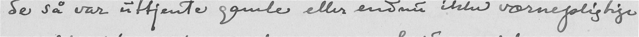de så var uttjente gamle eller endnu ikke værnepligtige
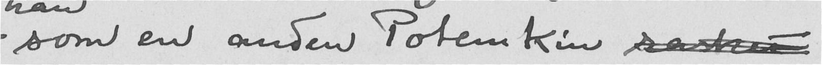som en anden Potemkin rasket
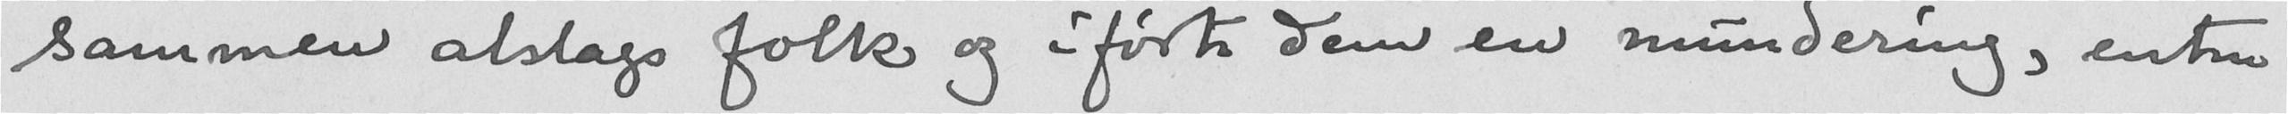sammen alslags folk og iførte dem en mundering, enten
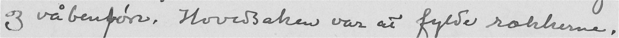og våbenføre. Hovedsaken var at fylde rækkerne.
